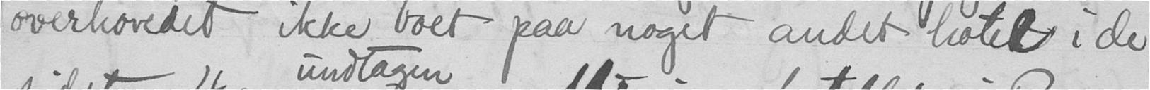overhovedet ikke boet paa noget andet hotel i de
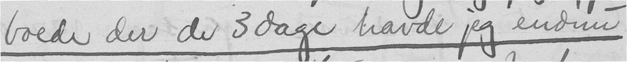boede der de 3 dage havde jeg endnu
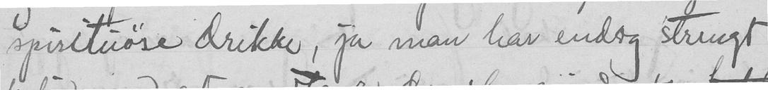spirituøse drikke, ja man har endog strengt
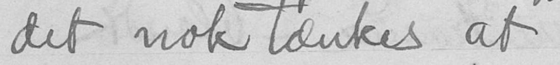det nok tænkes at
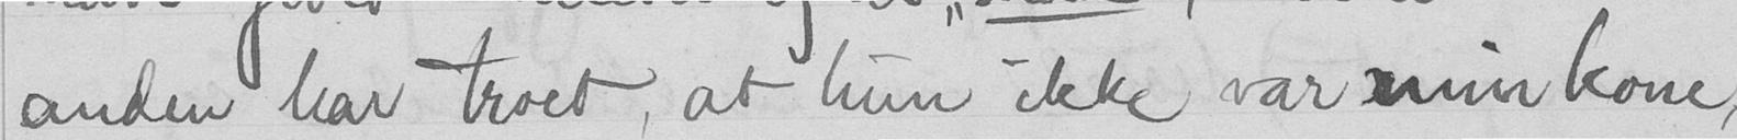anden har troet, at hun ikke var min kone,
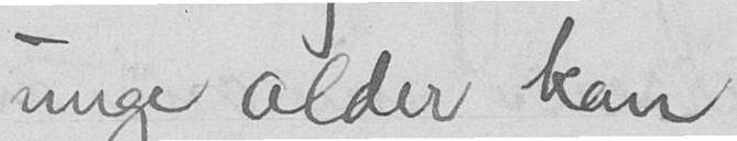unge alder kan
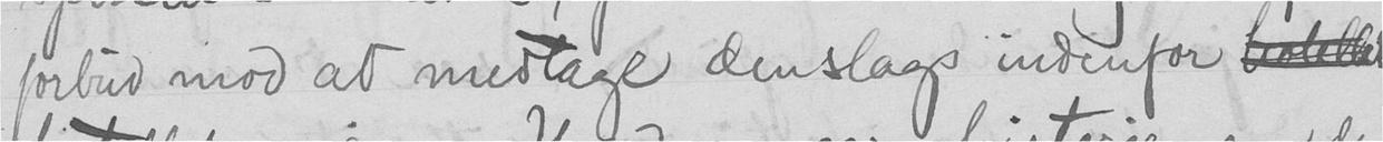forbud mod at medtage denslags indenfor
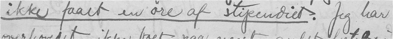ikke faaet en øre af stipendiet. Jeg har
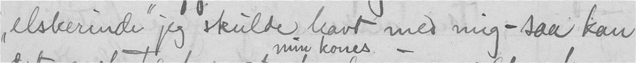"elskerinde" jeg skulde havt med mig - saa kan
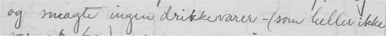og smagte ingen drikkevarer - (som heller ikke
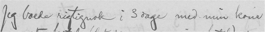Jeg boede rigtignok i 3 dage med min kone
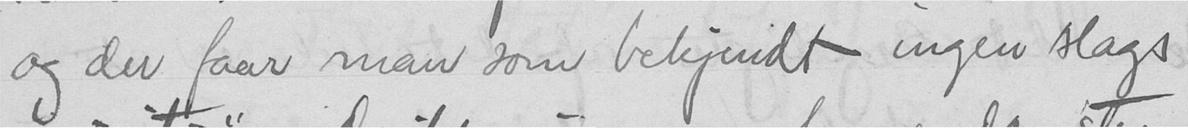og der faar man som bekjendt ingen slags
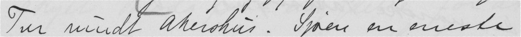Tur rundt Akershus. Sjøen en eneste
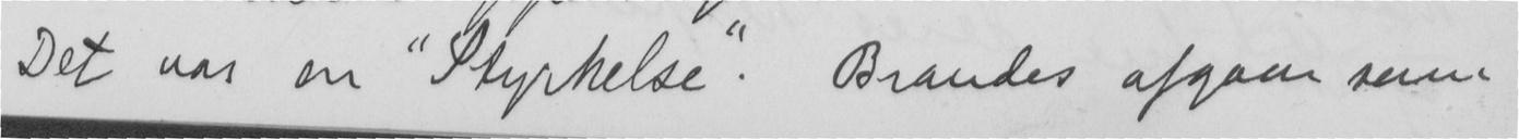Det var en "Styrkelse. Brandes afgaar som
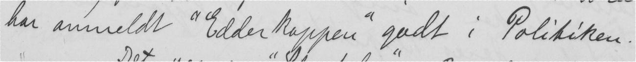har anmeldt "Edderkoppen" godt i Politiken.
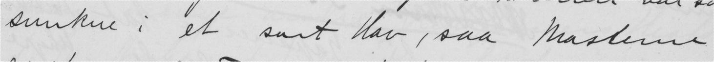sunkne i et sort Hav, saa Masterne
